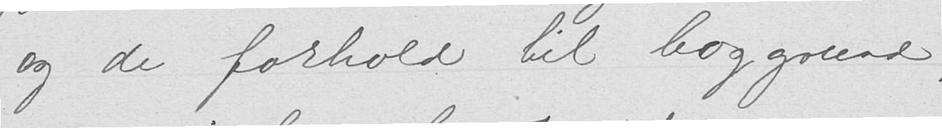og de forhold til baggrund,
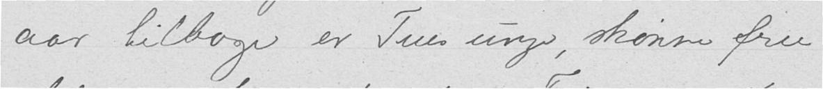aar tilbage er Tues unge, skønne fru
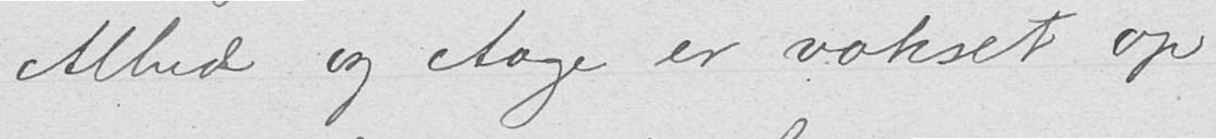Alhed og Aage er vokset op
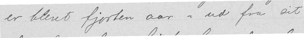er blevet fjorten aar - ud fra sit
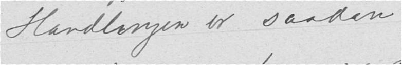Handlingen er saadan
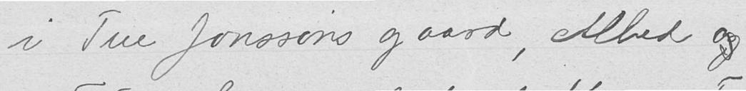i Tue Jonssøns gaard, Alhed og
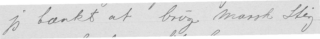jeg tænkt at bruge Marsk Stig
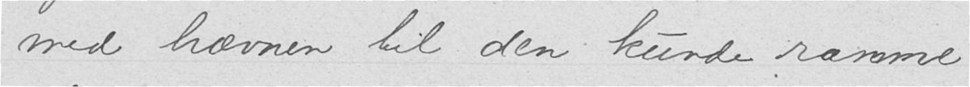med hævnen til den kunde ramme
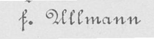f. Ullmann
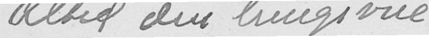Altid din hengivne
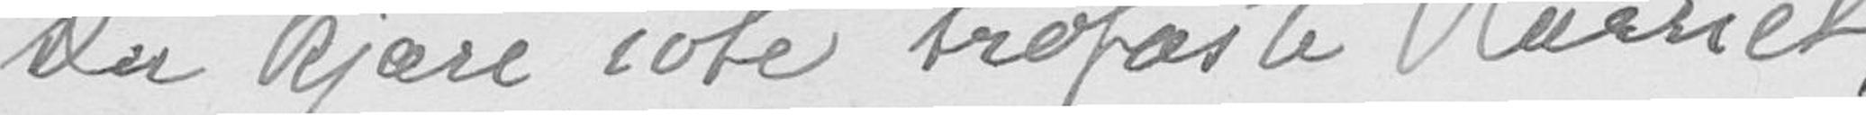Du kjære søte trofaste Harriet,
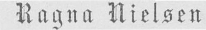Ragna Nielsen
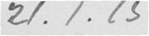21.1.15
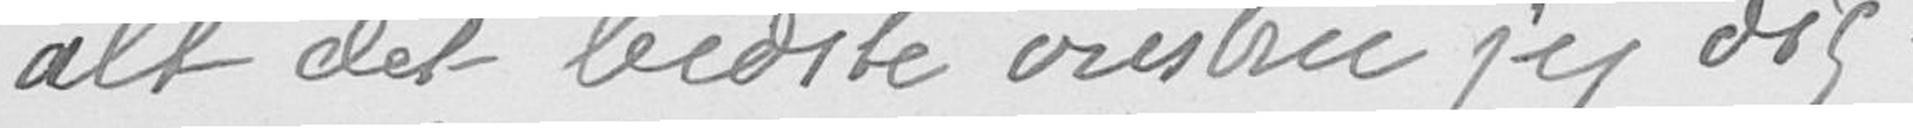alt det bedste ønsker jeg dig.
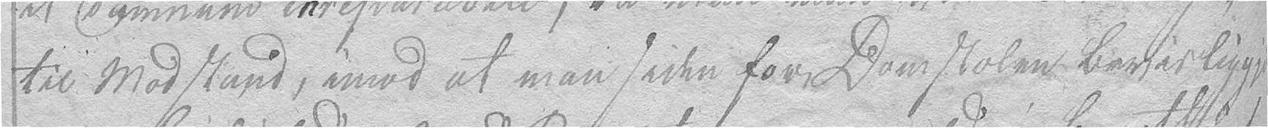til Modstand, imod at man siden for Domstolen bevisliggjør
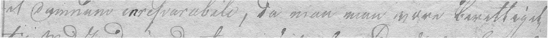et damnum irreparabile, da man maa være berettiget
